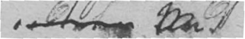og med
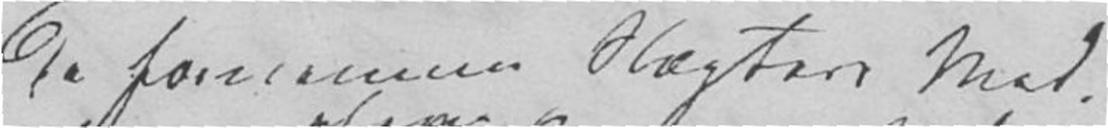de fornemme Slægters Med-
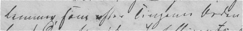lemmer, som efter Tingenes Orden
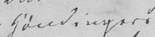Høvdingers
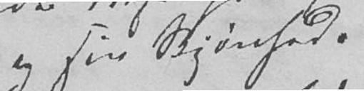og sin Skjønhed.
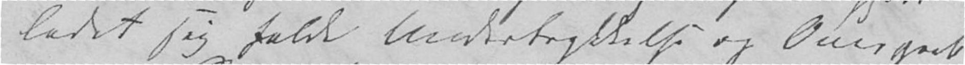ladet sig falde Undertrykkelse og Overgreb
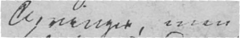Arvinger, men
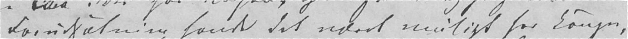Forudsætning havde det været muligt for Kongen,
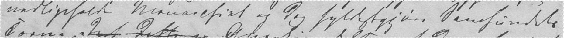vedligeholde Monarchiet og dog fyldestgjøre Samfundets
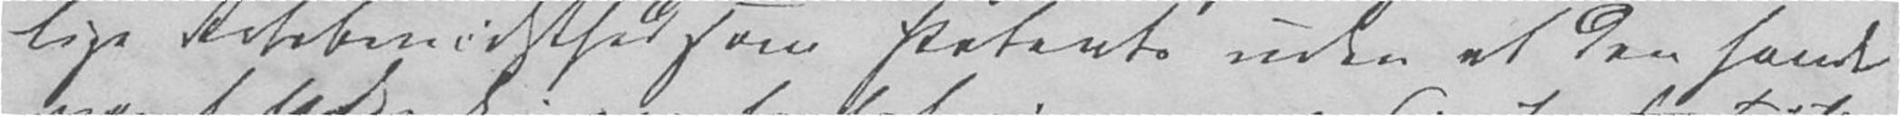lige Retsbevidsthed som Potents uden at den havde
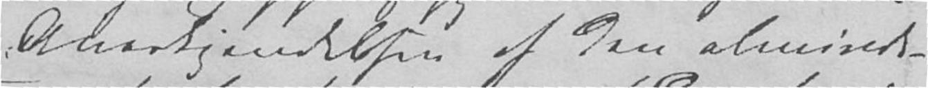Anerkjendelsen af den alminde-
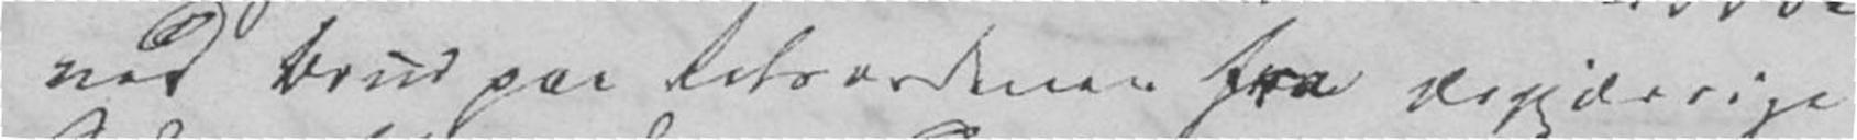ved Brud paa Retsordenen fra ærgjærrige
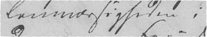Lovmæssigheden i
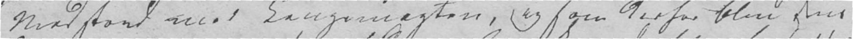Modstand mod Kongemagten, og som derfor blev dens
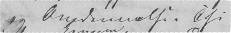og Omdannelse. Thi
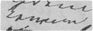kommer
